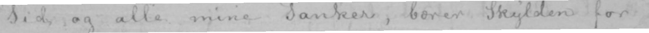Tid og alle mine Tanker, bærer Skylden for
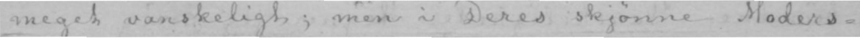meget vanskeligt; men i Deres skjønne Moders-
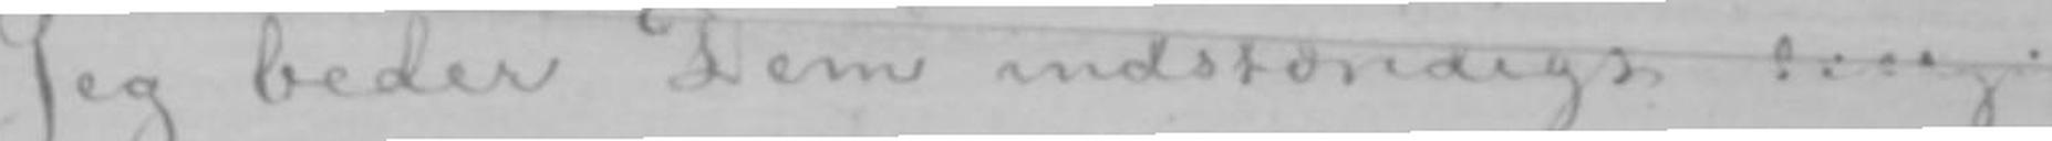Jeg beder Dem indstændigt tillgive
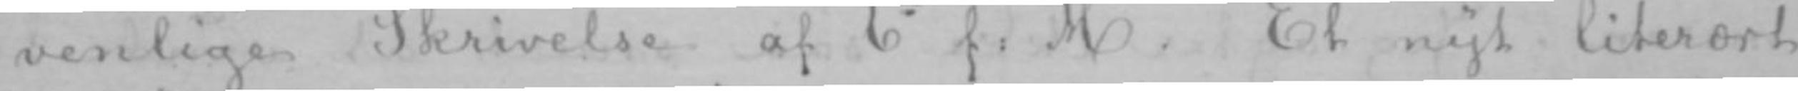venlige Skrivelse af 6te f: M: Et nyt literært
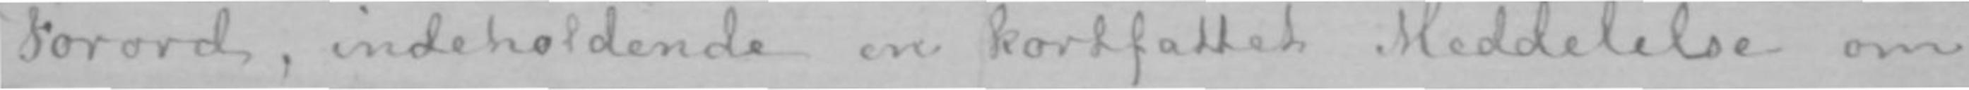Forord, indeholdende en kortfattet Meddelelse om
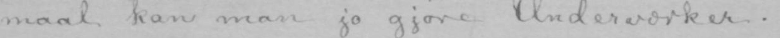maal kan man jo gjøre Underværker.-
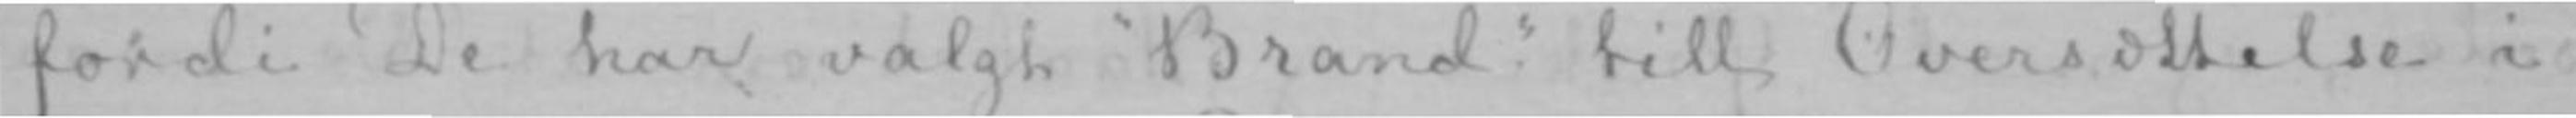fordi De har valgt «Brand» till Oversættelse i
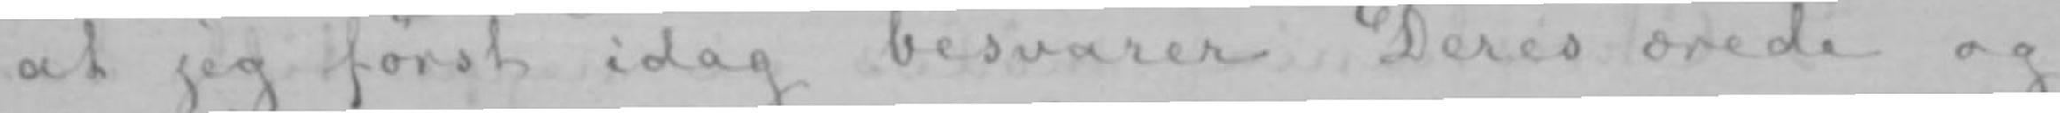at jeg først idag besvarer Deres ærede og
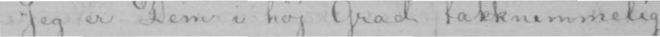Jeg er Dem i høj Grad takknemmelig
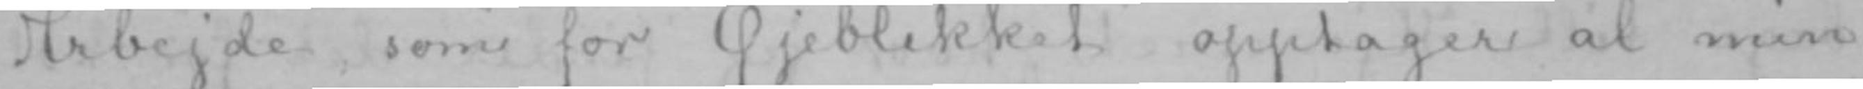Arbejde, som for Øjeblikket opptager al min
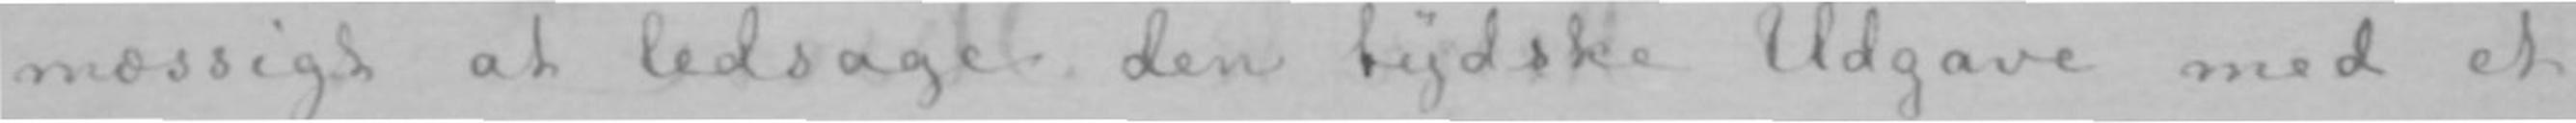mæssigt at ledsage den tydske Udgave med et
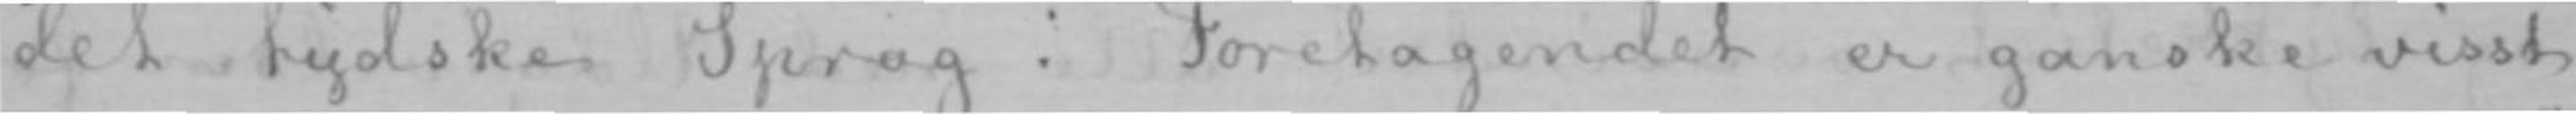det tydske Sprog. Foretagendet er ganske visst
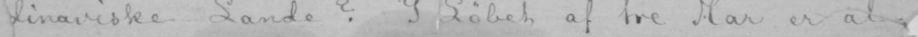dinaviske Lande? I Løbet af tre Aar er al-
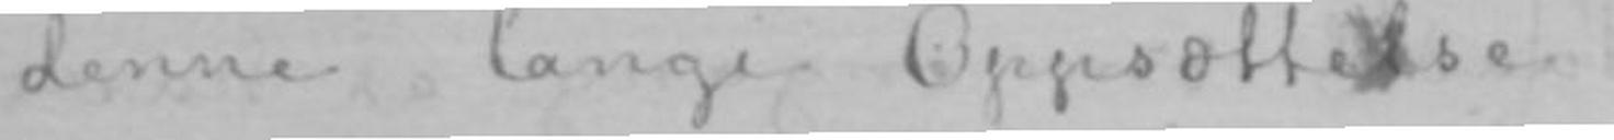denne lange Oppsættelse.
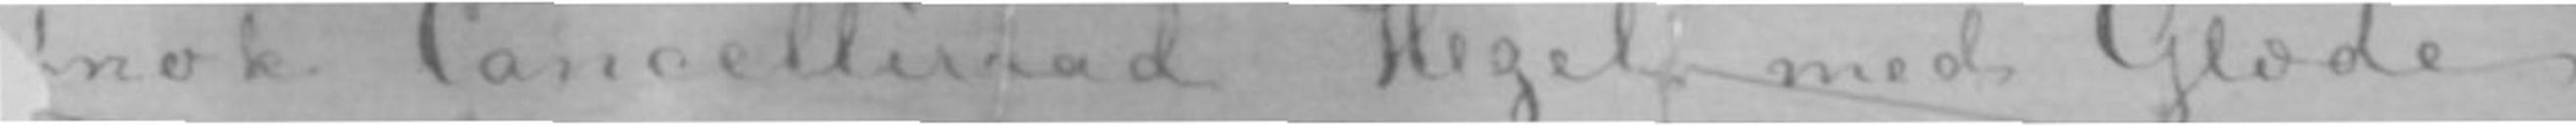nok Cancelliraad Hegel med Glæde
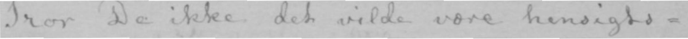Tror De ikke det vilde være hensigts-
